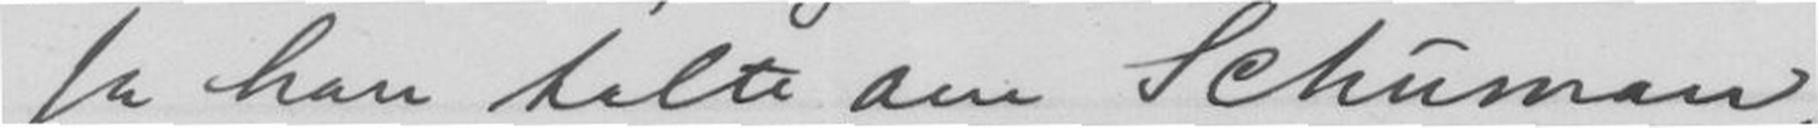Ja han talte om Schuman.
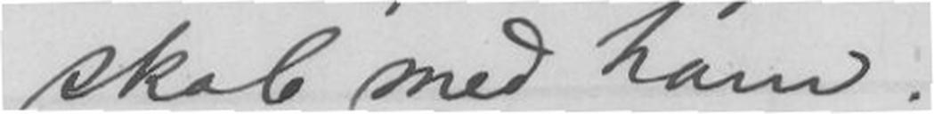skab med ham.
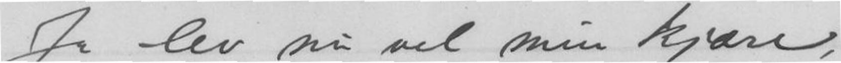Ja lev nu vel min kjære,
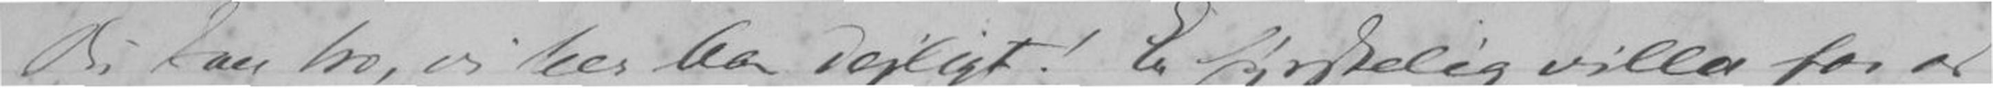Du kan tro, vi her har Dejligt! En fyrstelig villa for os
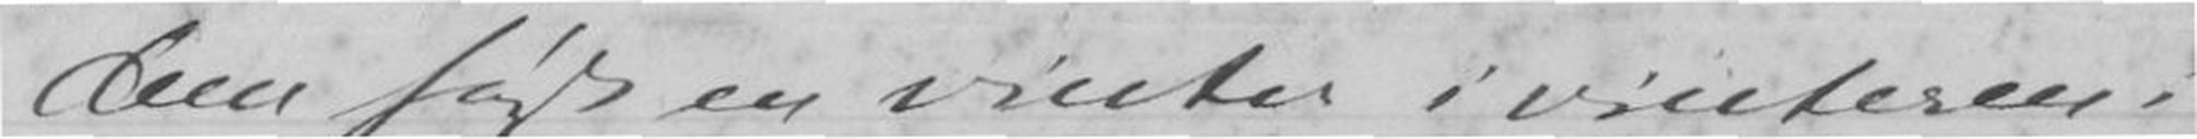Men først en vinter i vinteren!
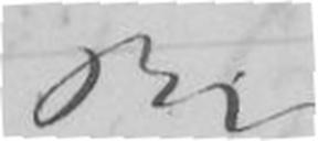Bj
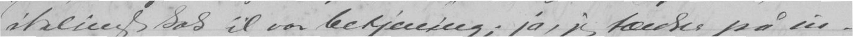italiensk kok til vor betjening; ja, jeg tænker på in-
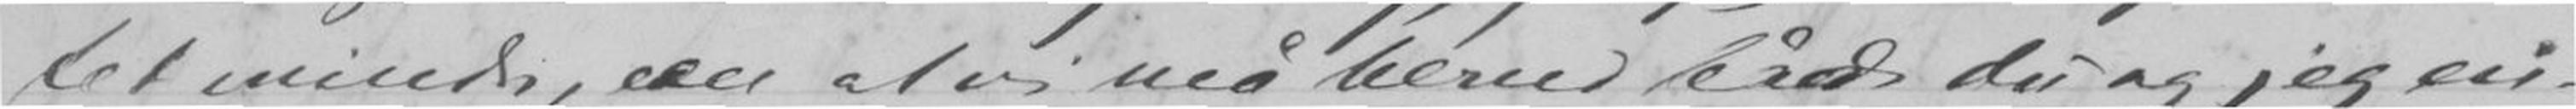tet mindre, æn at vi må herned både du og jeg en-
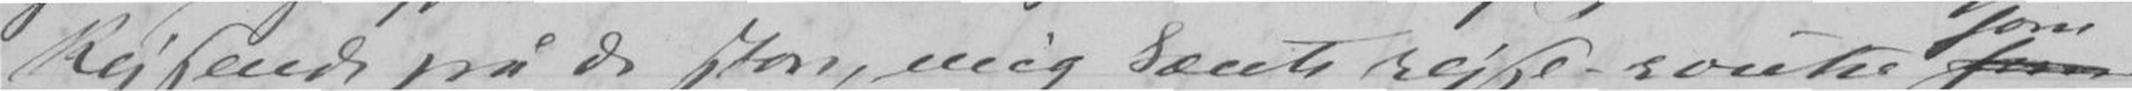Rejsende på de store, mig kænte rejse-router som
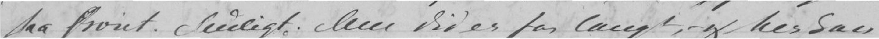saa skønt. Muligt. Men did er for langt, og her kan
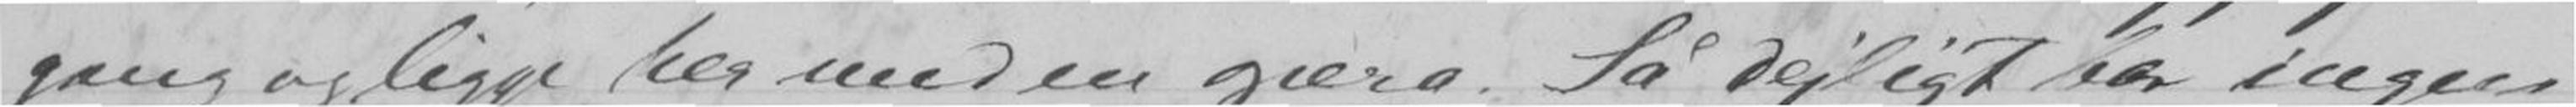gang og ligge her med en opera, Så dejligt bor ingen
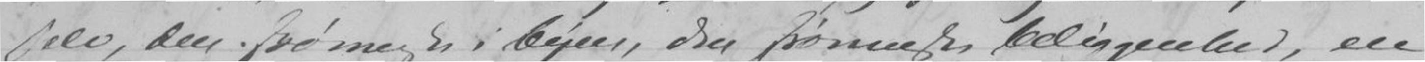selv, den skønneste i byen, den skønneste beligenhed, en
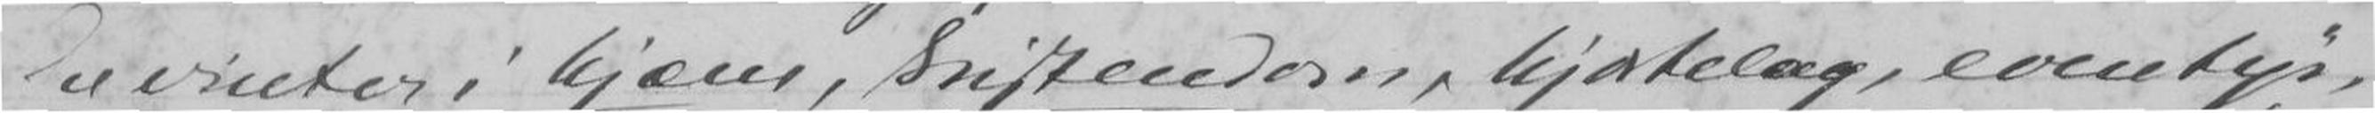Du vinter i hjæm, kristendom, hjærtelag, eventyr,
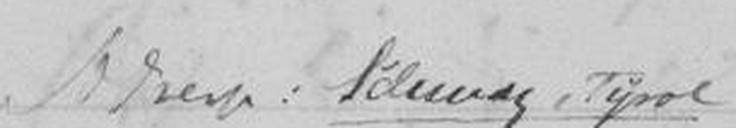Adresse: Schwaz, Tyrol
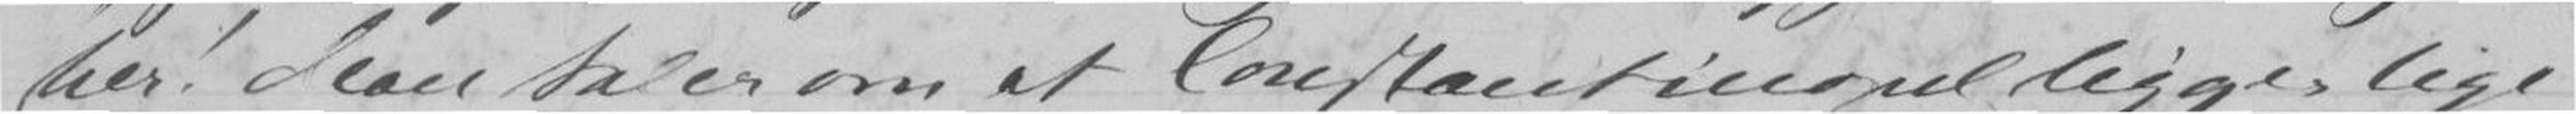her! Man taler om at Constantinopel ligger lige
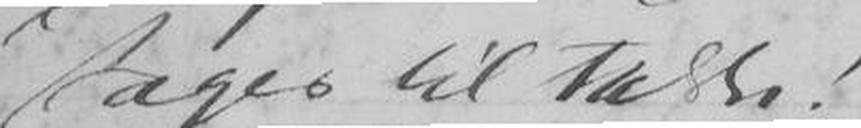tages til takke!
